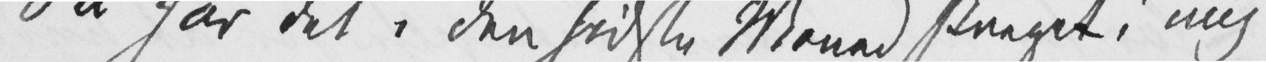Så har det i den sidste Måned skreget i mig
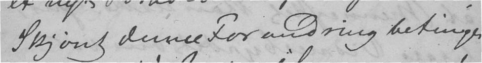Skjønt denne Forundring betinges
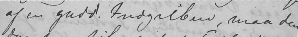af en gudd. Indgriben, maa den
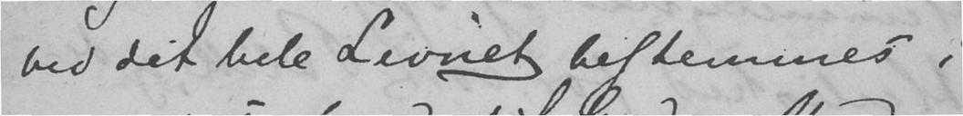ved det hele Levnet bestemmes i
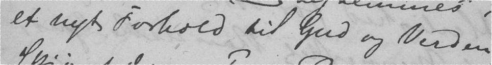et nyt Forhold til Gud og Verden,
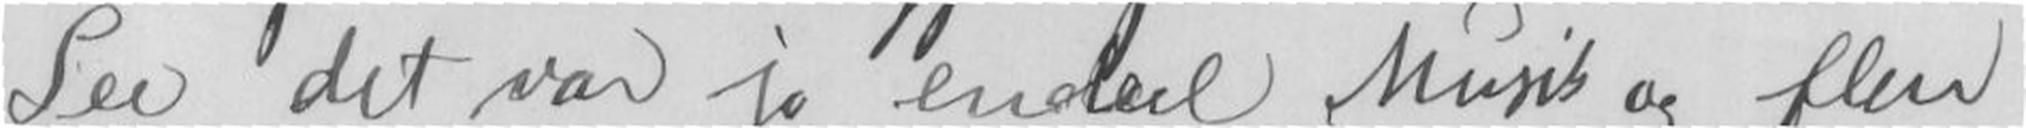See det var jo endel Musik og flere
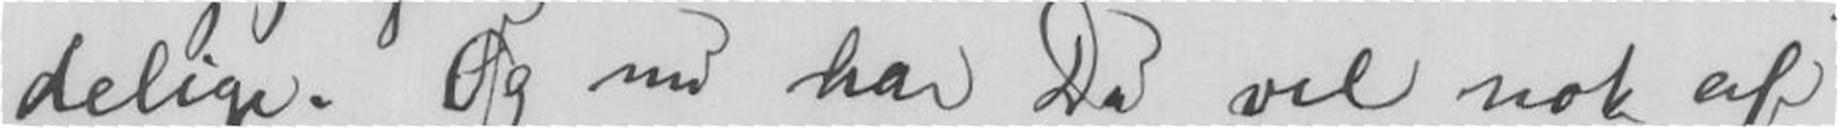delige. Og nu har Du vel nok af
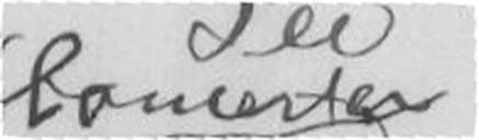Concerter
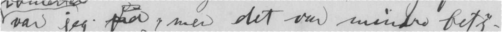var jeg paa, men det var mindre bety-
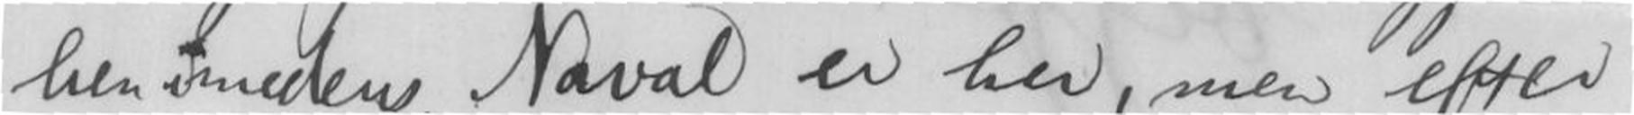hen imedens. Naval er her, men efter
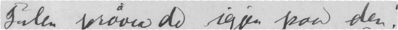Julen prøver de igjen paa den.
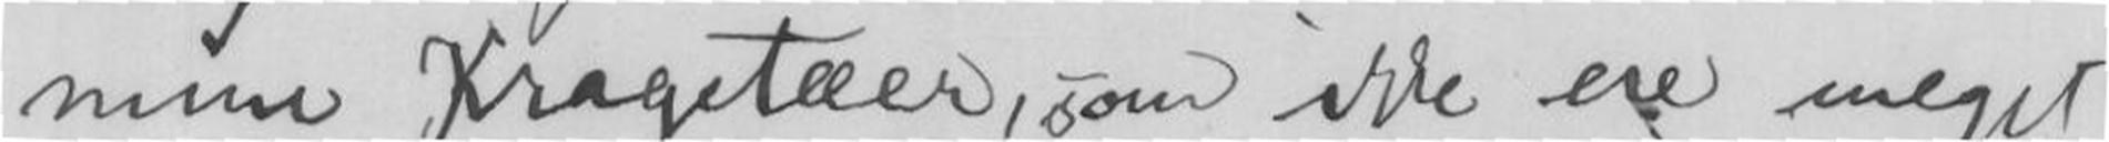mine Krogetæer, som ikke ere meget
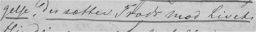gelse, der sætter Trods mod Livets
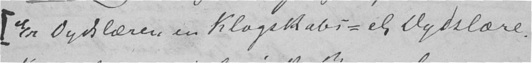[Er Dydslæren en Klogskabs- el Dydslære.
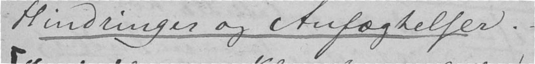Hindringer og Anfægtelser.
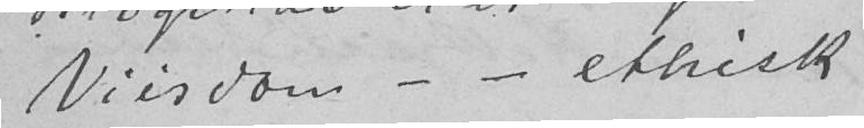Viisdom - - ethisk
